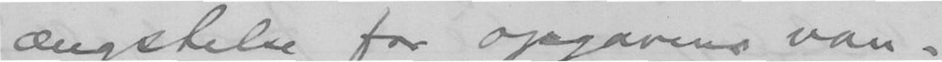ængstelse for opgavens van-
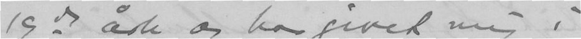19 de årh og har givet mig i
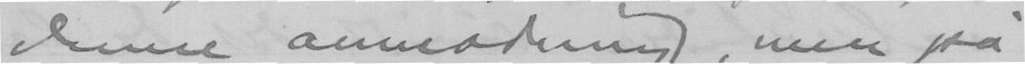denne anmodning, men på
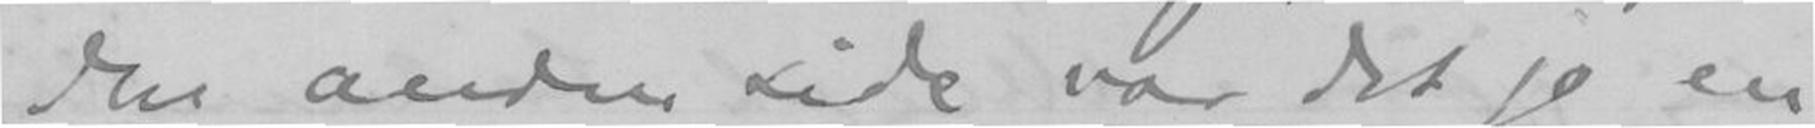den anden side var det jo en
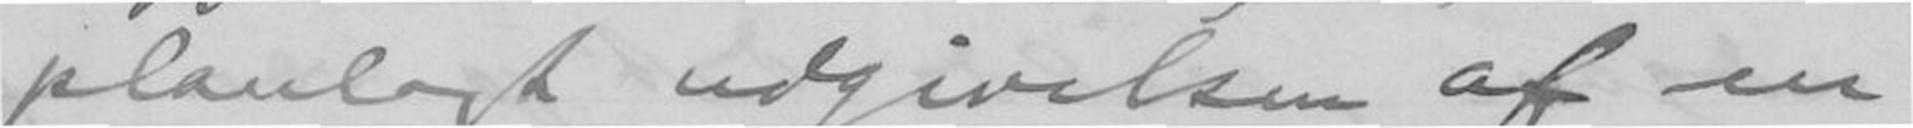planlagt udgivelsen af en
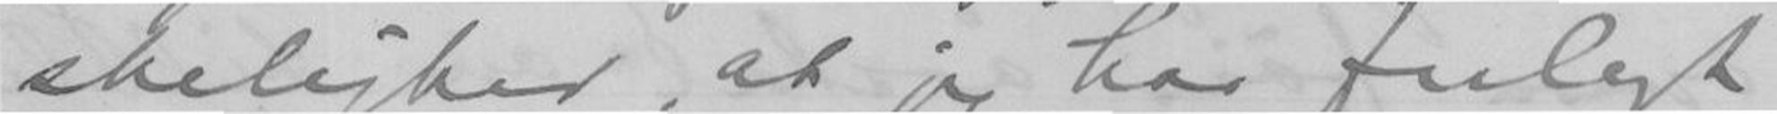skelighed, at jeg har fulgt
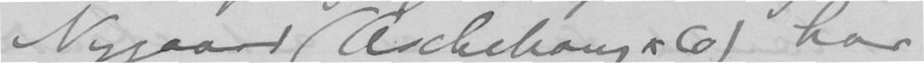Nygaard (Aschehaug & Co) har
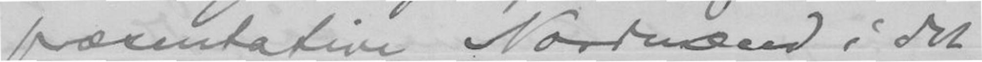præsentative Nordmænd i det
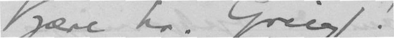Kjære hr. Grieg!
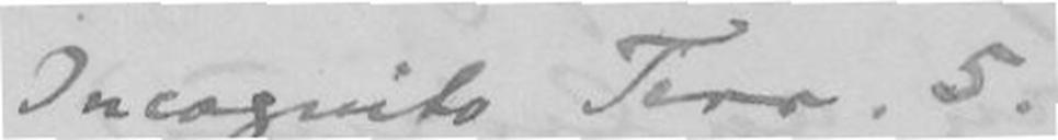Incognito Terr. 5.
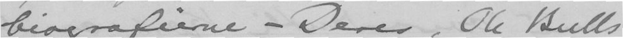biografierne - Deres, Ole Bulls,
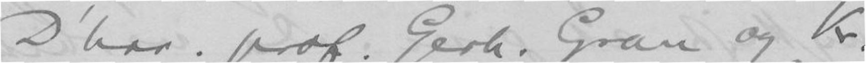D'hrr. prof. Gerh. Gran og Kr.
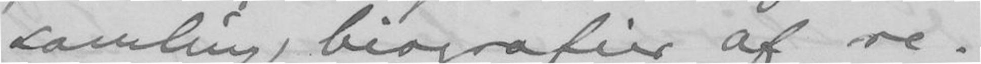samling biografier af re-
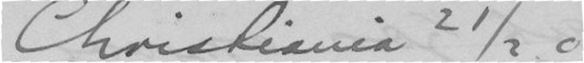Christiania 21/2.01
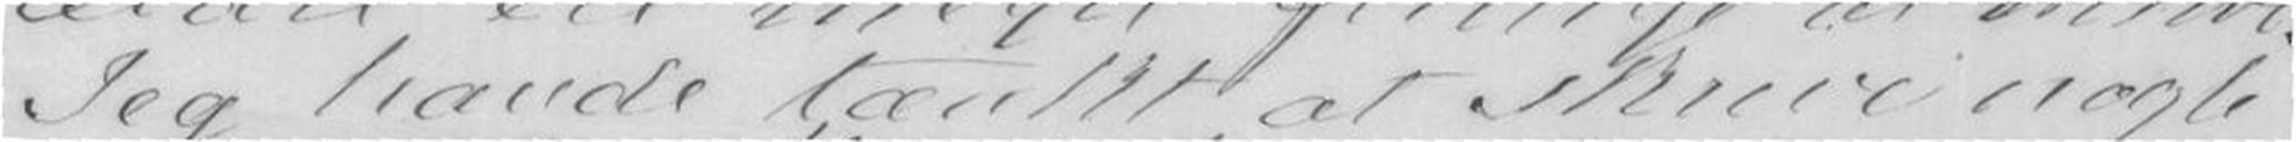Jeg havde tænkt at skrive nogle
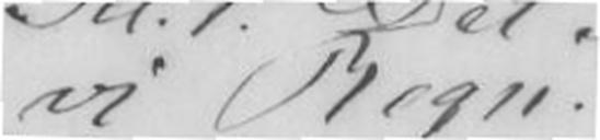vi Regn.
